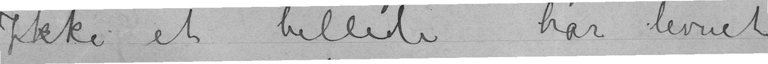Ikke et billede har levnet
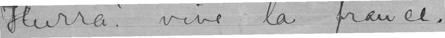Hurra! vive la france! –
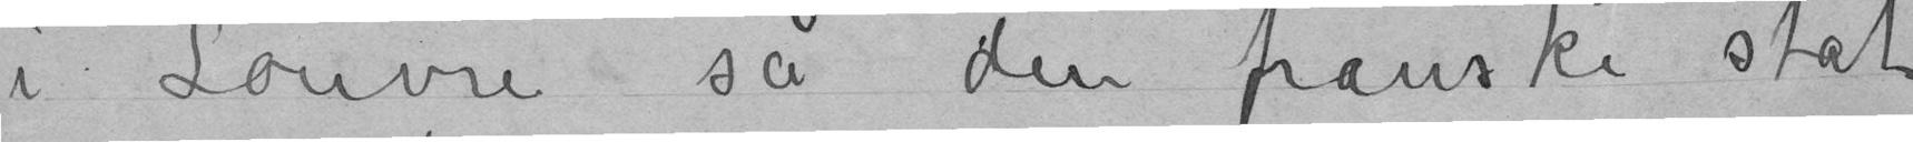i Louvre så den franske stat
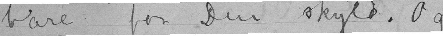bare for Din skyld. Og
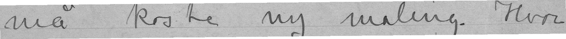må koste ny maling. Hvor
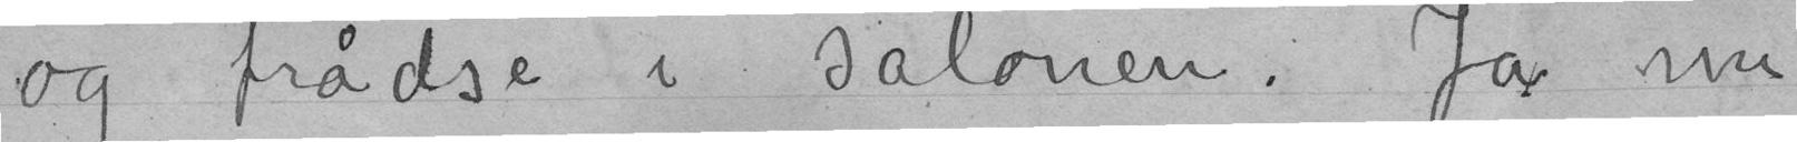og frådse i salonen. Ja nu
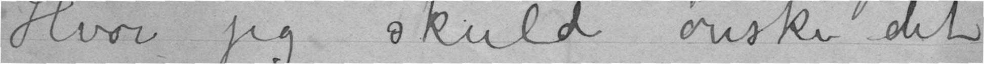Hvor jeg skulde ønske det
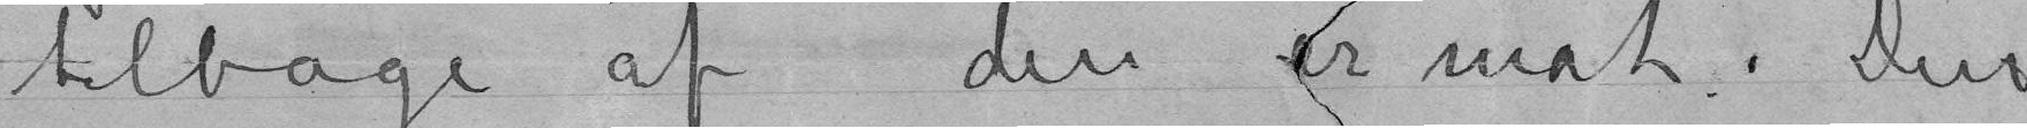tilbage af den er mat. Den
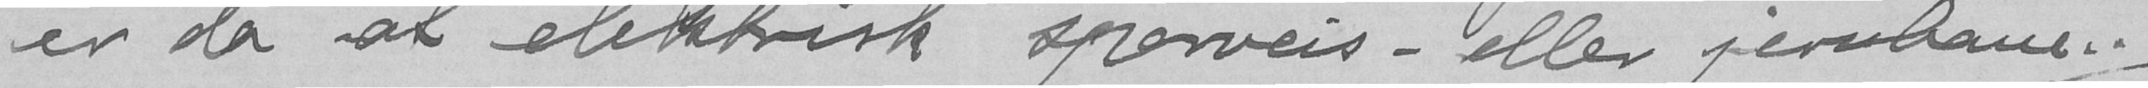er da at elektrisk sporveis- eller jernbane-
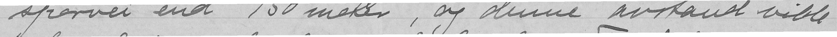sporvei end 150 meter, og denne avstand ville
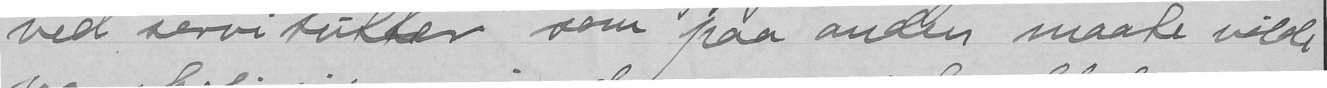ved servitutter som paa anden maate vilde
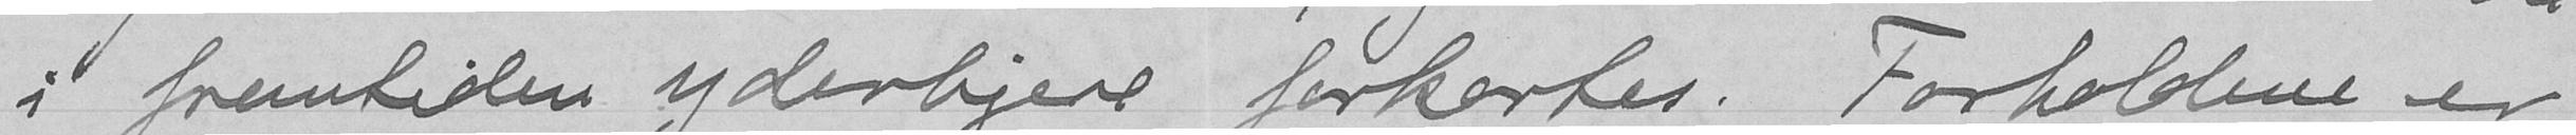i fremtiden yderligere forkortes. Forholdene er
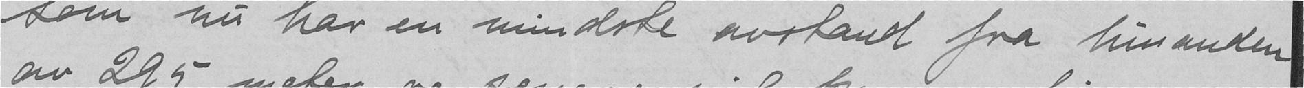som nu har en mindste avstand fra hinanden
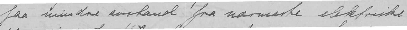faa mindre avstand fra nærmeste elektriske
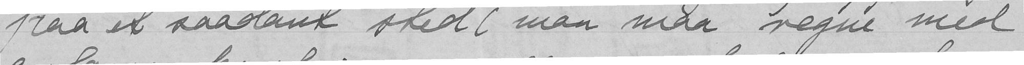paa et saadant sted (man maa regne med
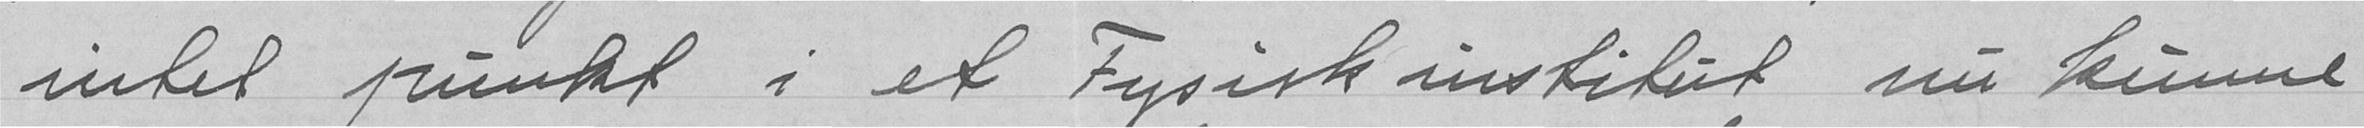intet punkt i et Fysikinstitut nu kunne
